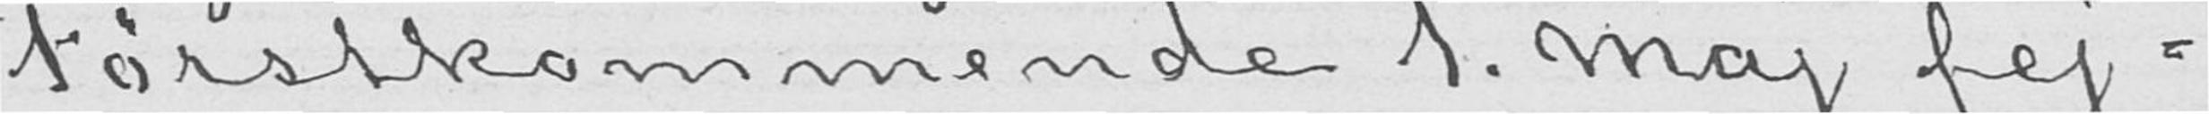Førstkommende 1. maj fej-
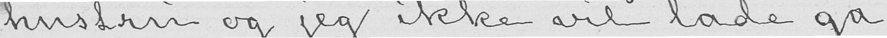hustru og jeg ikke vil lade gå
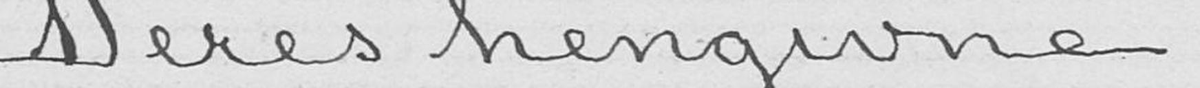Deres hengivne
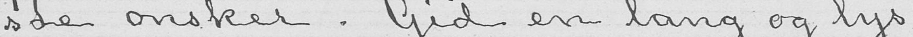ste ønsker. Gid en lang og lys
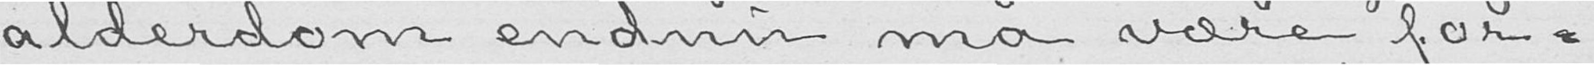alderdom endnu må være for-
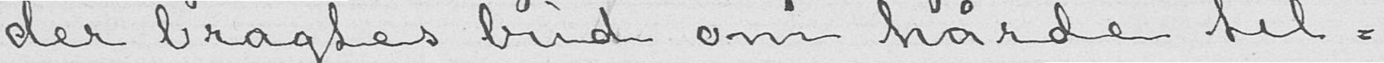der bragtes bud om hårde til-
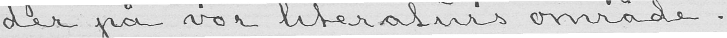der på vor literaturs område.
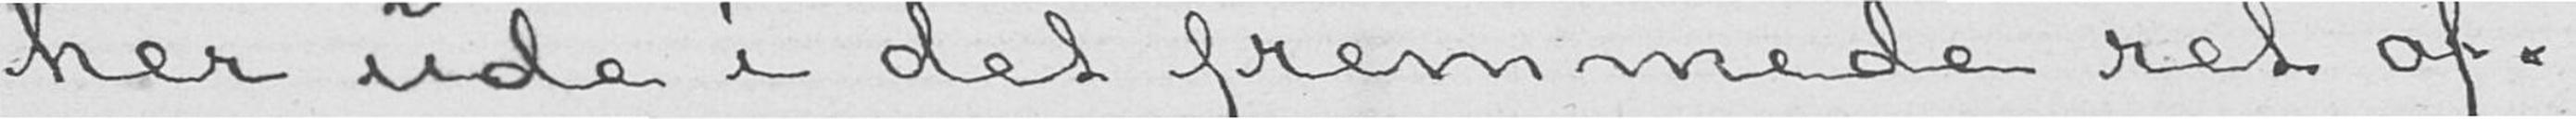her ude i det fremmede ret of-
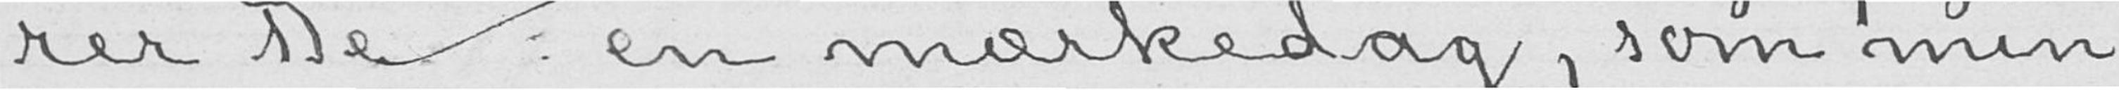rer De en mærkedag, som min
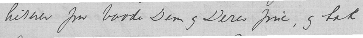hilsener fra baade Dem og Deres frue, og tak
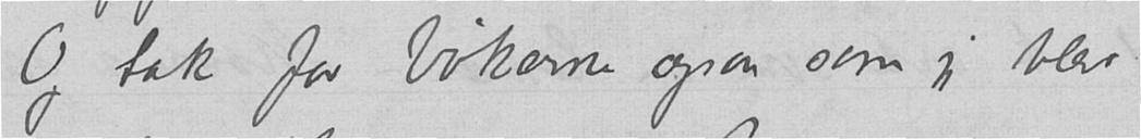Og tak for bøkerne ogsaa som jeg blev
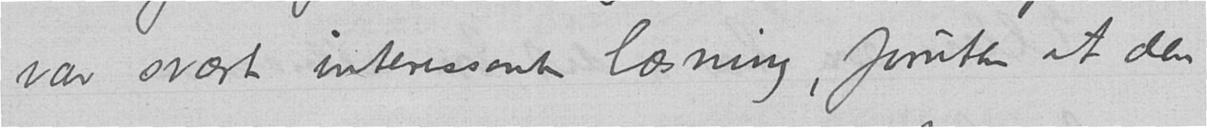var svært interessant læsning, foruten at den
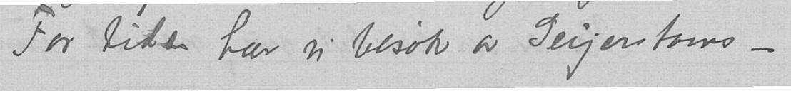For tiden har vi besøk av Geijerstams –
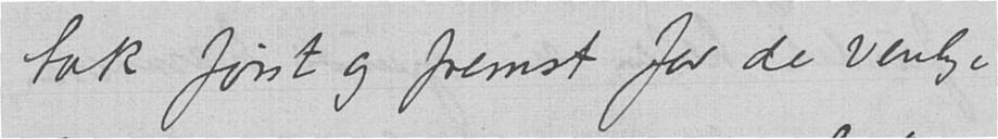tak først og fremst for de venlige
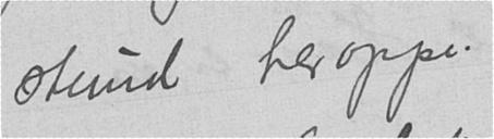stund heroppe.
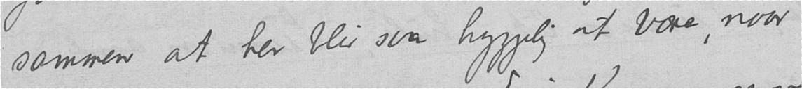sammen at her blir saa hyggelig at være naar
